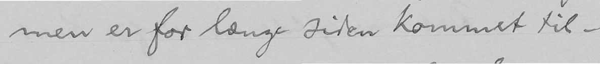men er for længe siden kommet til-
